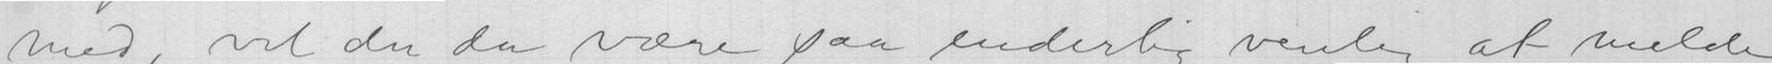med, vil du da være saa inderlig venlig at melde
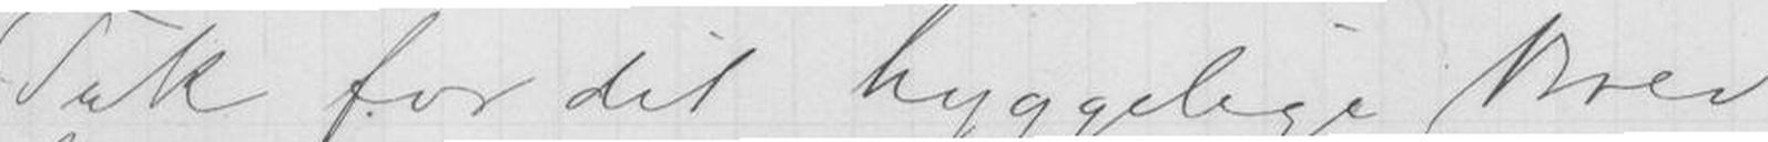Tak for dit hyggelige Brev
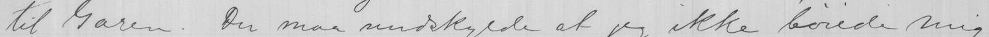til Garen. Du maa undskylde at jeg ikke bøiede mig
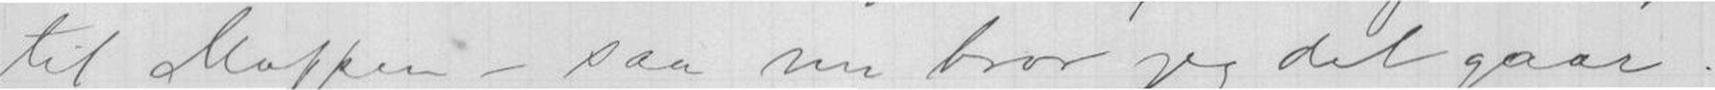til Mappen - saa nu tror jeg det gaar.
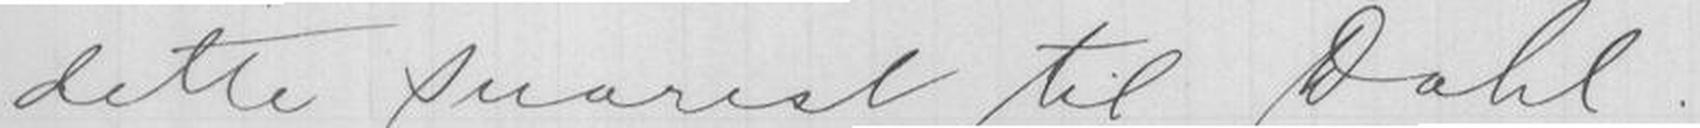dette snarest til Dahl.
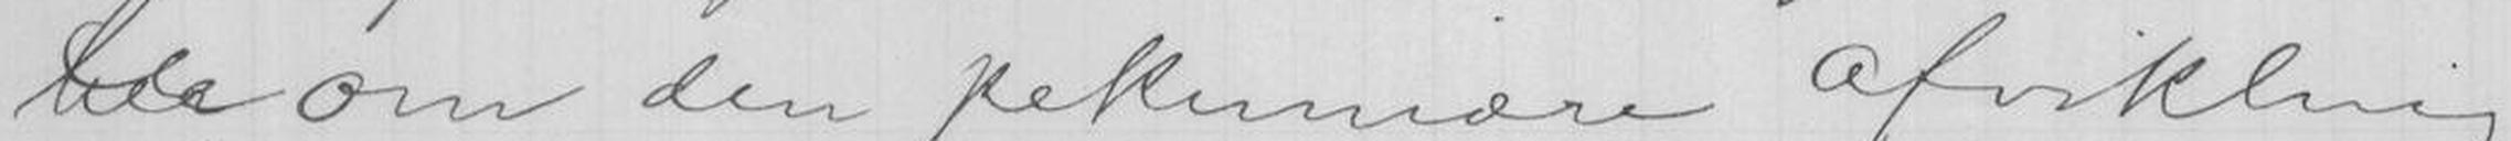om den pekuniære Afvikling
